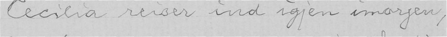Cecilia reiser ind igjen imorgen,
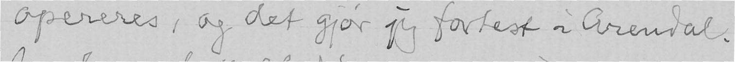opereres, og det gjør jeg fortest i Arendal.
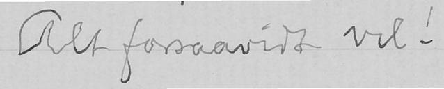Alt forsaavidt vel!
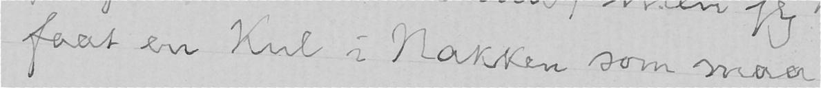faat en Kul i Nakken som maa
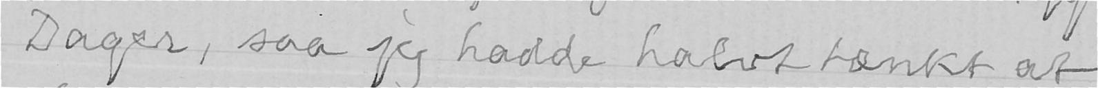Dager, saa jeg hadde halvt tænkt at
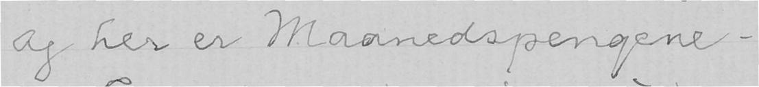og her er Maanedspengene -
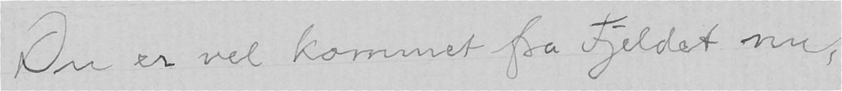Du er vel kommet fra Fjeldet nu,
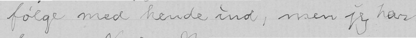følge med hende ind, men jeg har
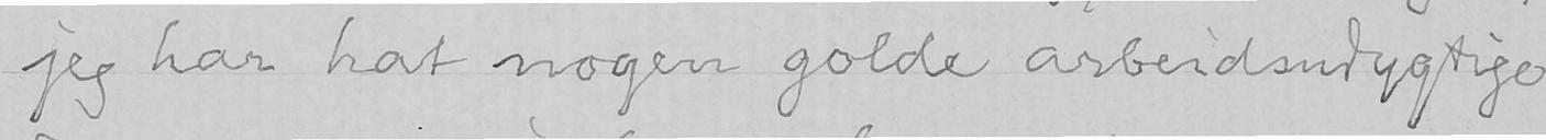jeg har hat nogen golde arbeidsudygtige
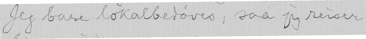Jeg bare lokalbedøves, saa jeg reiser
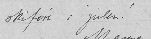skiføre i julen!
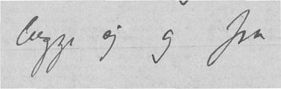legge sig og fra
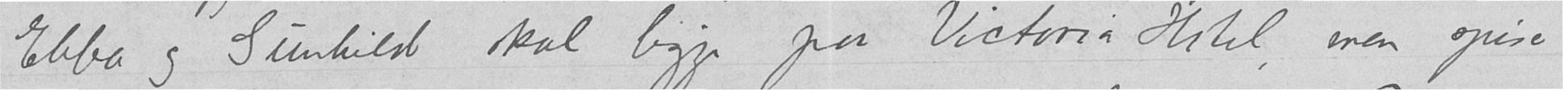Ebba og Gunhild skal ligge paa Victoria Hotel, men spiser
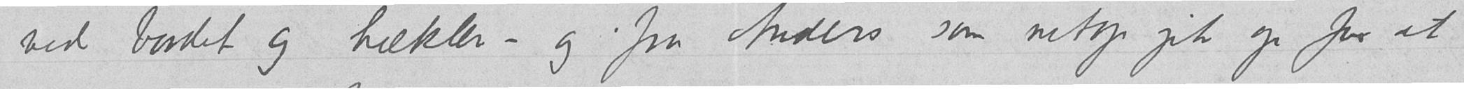ved bordet og hækler – og fra Anders som netop gik op for at
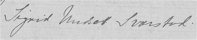Sigrid Undset Svarstad.
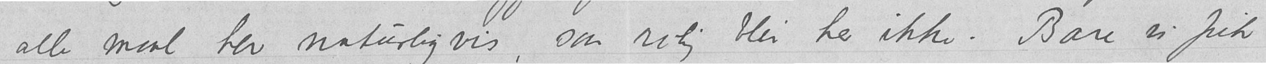alle maal her naturligvis, saa rolig blir her ikke. Bare vi fik
